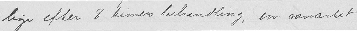lige efter 8 timers behandling, en vanartet
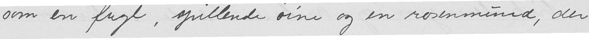som en fugl, spillende øine og en rosenmund, der
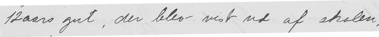12aars gut, der blev vist ud af skolen,
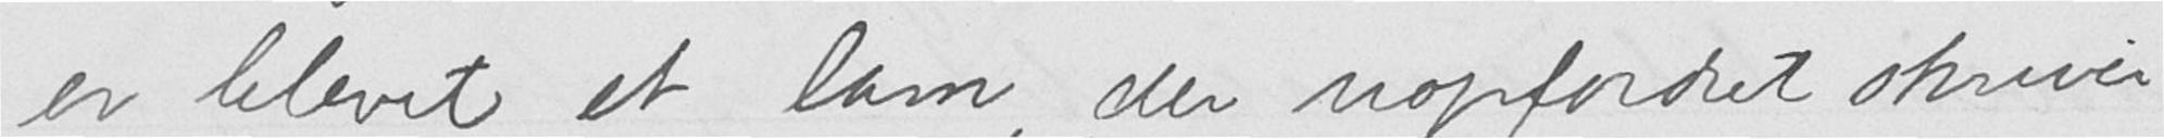er blevet et lam, der uopfordret skriver
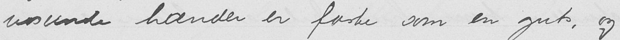udseende hænder er faste som en guts, og
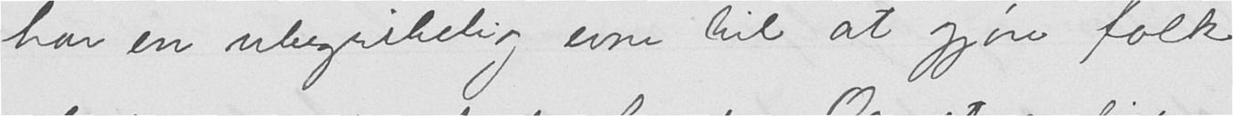har en ubegribelig evne til at gjøre folk
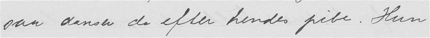saa danser de efter hendes pibe. Hun
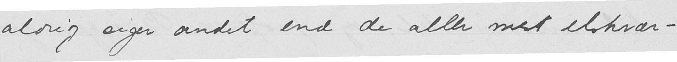aldrig siger andet end de aller mest elskvær-
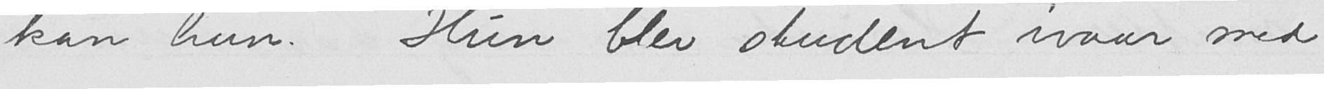kan hun. Hun blev student ivaar med
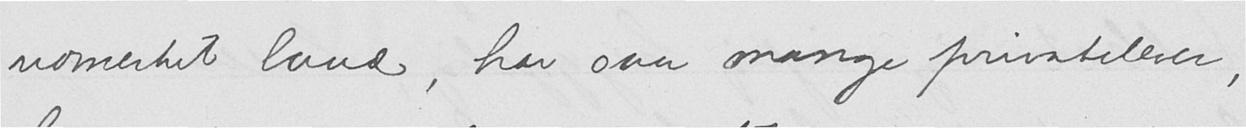udmerket laud, har saa mange privatelever,
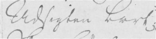Udsigten bort;
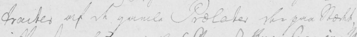traiter af de gamle Prælater der paa Stædet
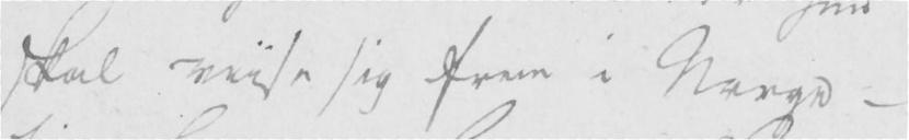skal viise sig frem i Norge -
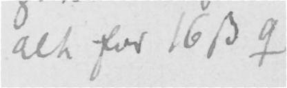alt for 16 s q
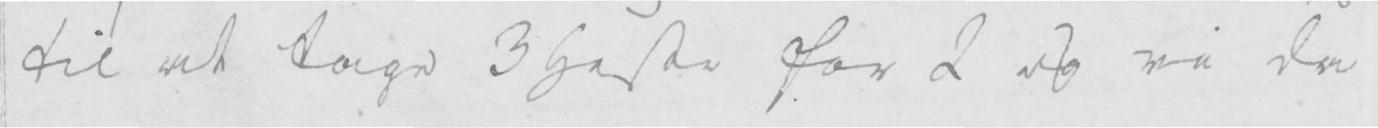til at tage 3 Heste for 2 og vi da
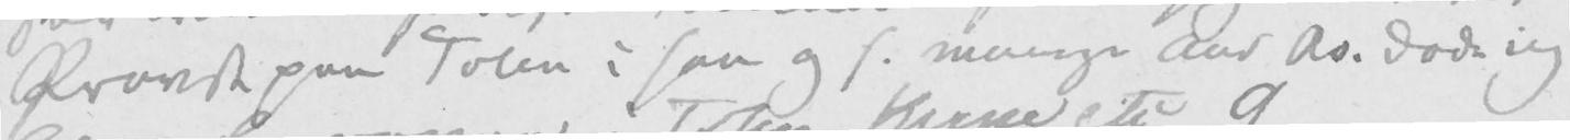Provst paa Toten i saa og s. mange aar As. døde ig
